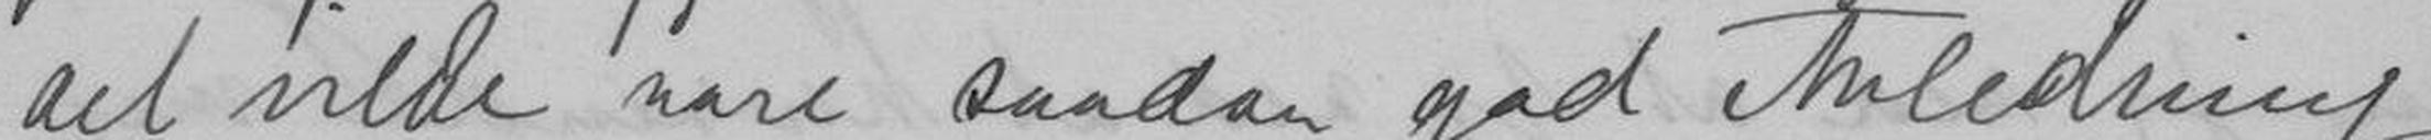det vilde være saadan god Anledning
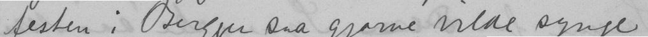festen i Bergen saa gjærne vilde synge
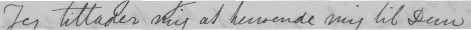Jeg tillader mig at henvende mig til Dem
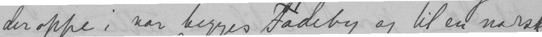der oppe i vor begges Fødeby og til en norsk
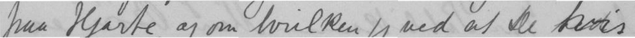paa Hjærte, og om hvilken jeg ved at De hvis
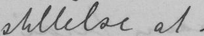stillelse at
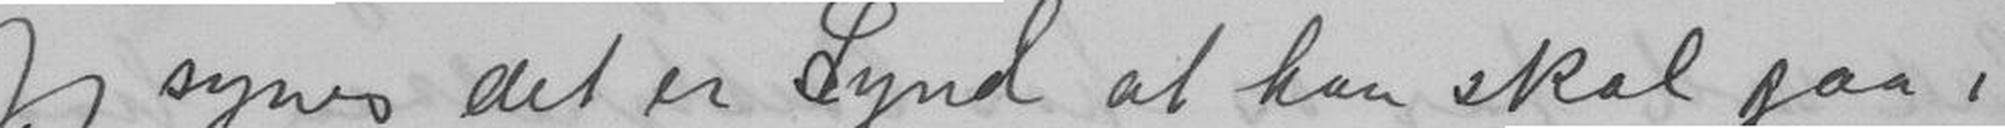Jeg synes det er Synd at han skal gaa i
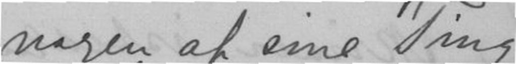nogen af sine Ting
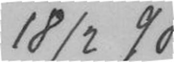18/2 98.
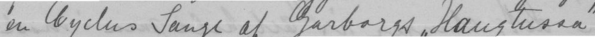en Cyclus Sange af Garborgs "Haugtussa"
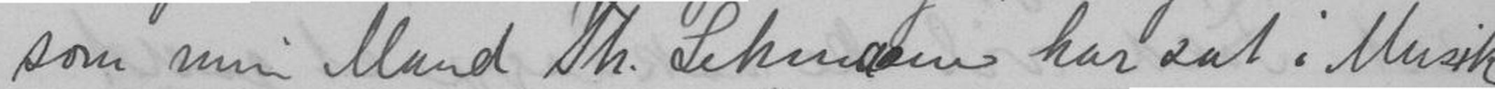som min Mand Th. Lehmann har sat i Musik
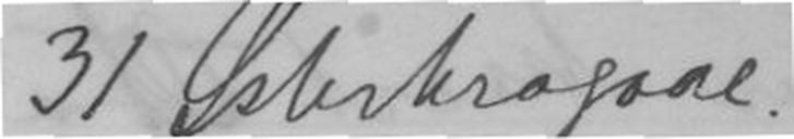31 Østerbrogade.
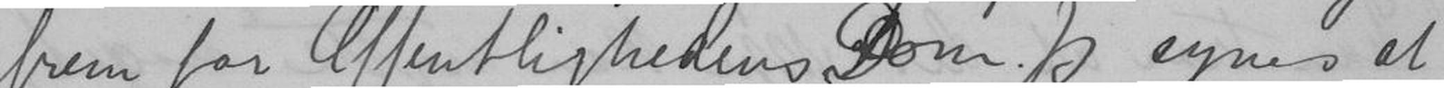frem for Offentlighedens Dom. Jeg synes at
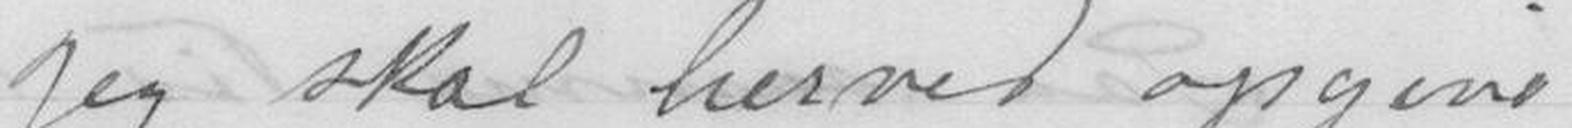Jeg skal herved opgive
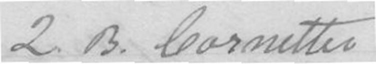2. B.Cornetter
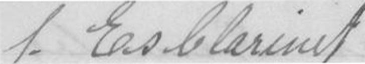1. Es Clarinet
